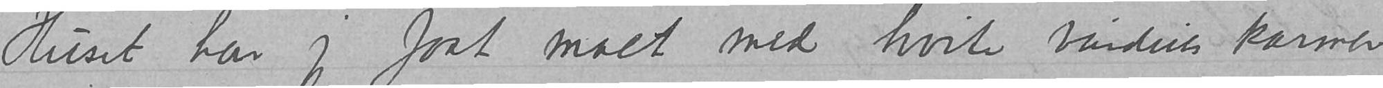Huset har jeg faat malt med hvite vindus karmer
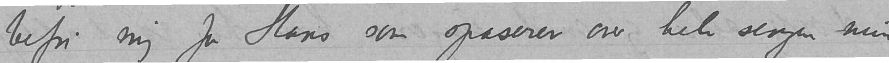og befri mig for Hans som spaserer over hele sengen min
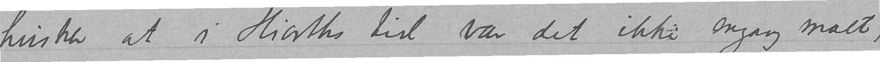husker at i Hiorths tid var det ikke engang malt,
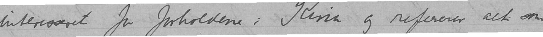interesseret for forholdene i Kina og refererer alt som
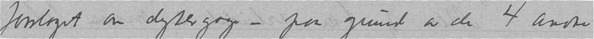forslaget om digtergage – paa grund av de 4 andre
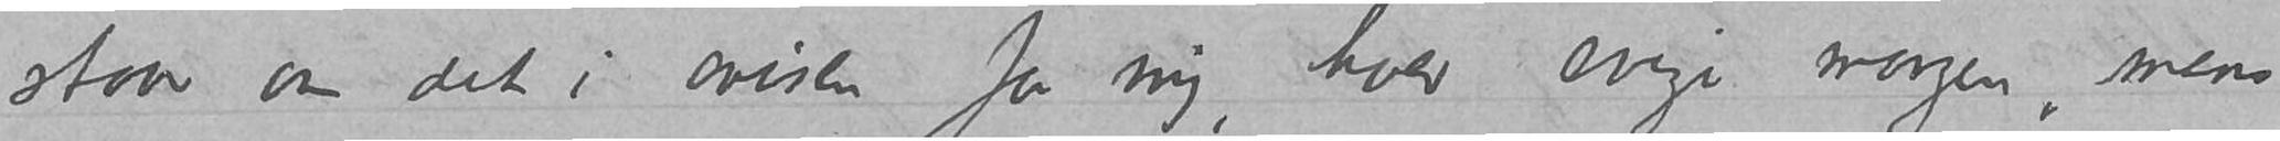staar om det i avisen for mig, hver evige morgen, mens
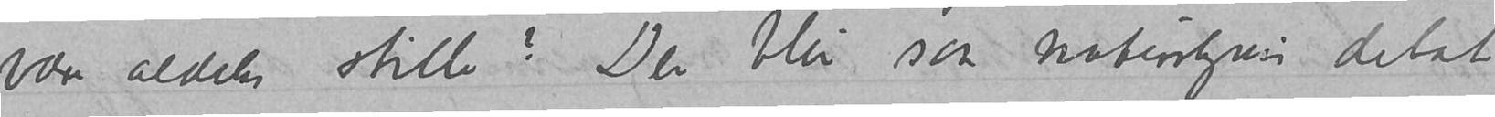være aldeles stille?  Der blir saa naturligvis debat
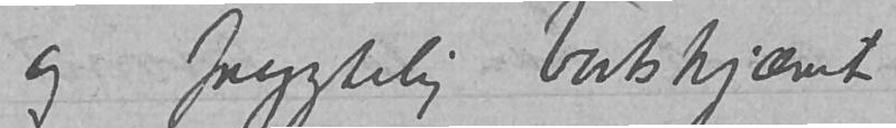og frygtelig bortskjæmt.
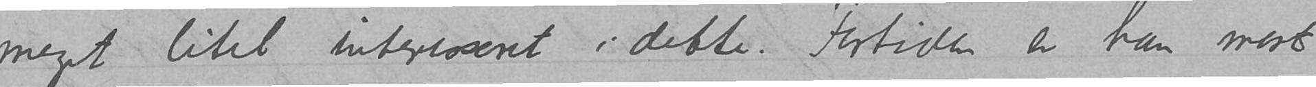meget litet interesseret i dette.  Fortiden er han mest
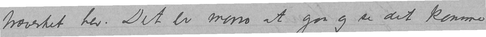træverket her. Det er morro at gaa og se det kommer
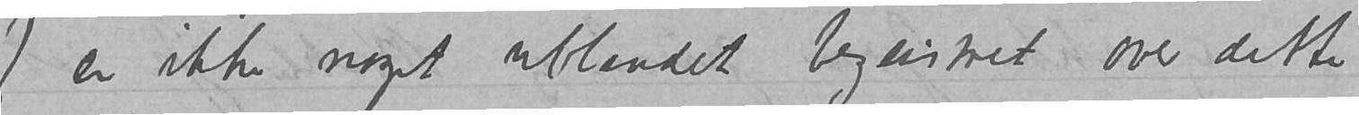Jeg er ikke noget ublandet begeistret over dette
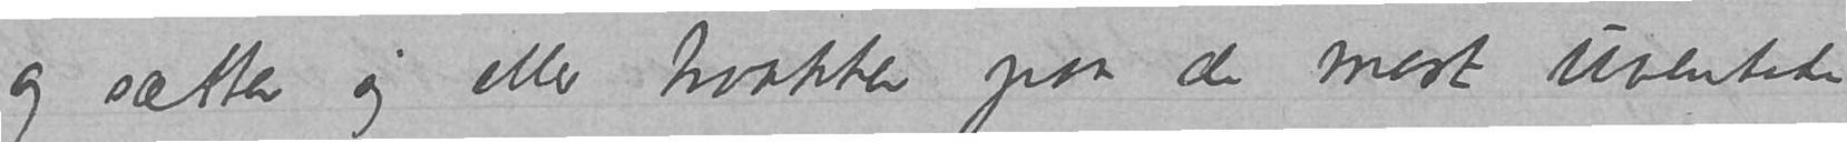og sætter sig eller krabber traakker paa de mest uventede
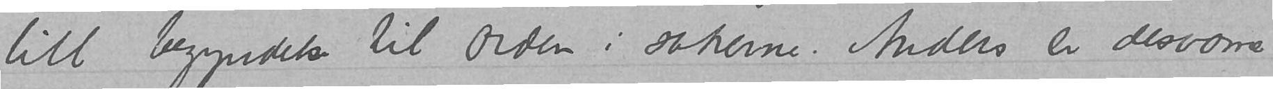litt begyndelse til orden i sakerne.  Anders er desværre
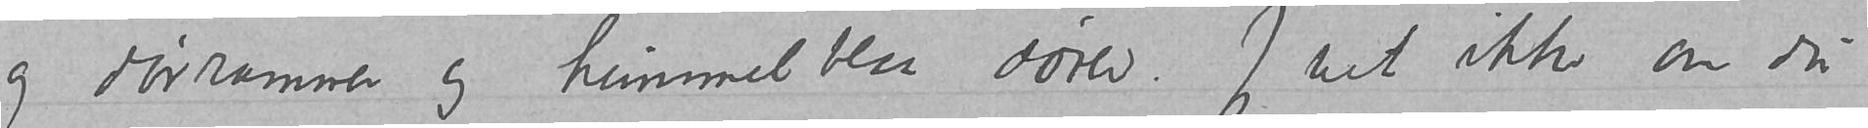og dørrammer og himmelblaa dører. Jeg vet ikke om du
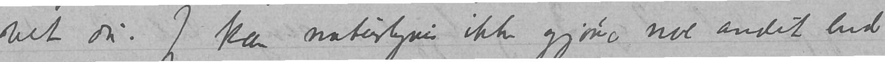vet du. Jeg kan naturligvis ikke gjøre noe andet end
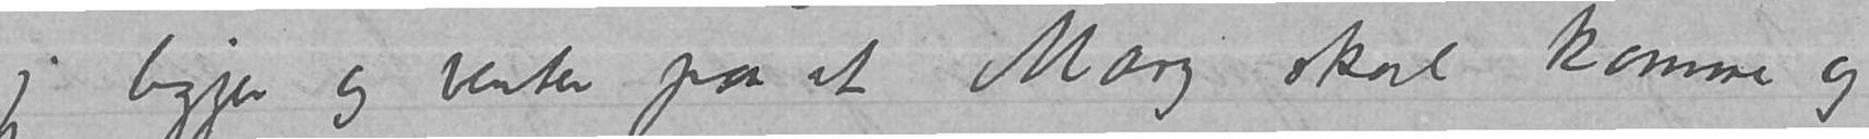jeg ligger og venter paa at Mary skal komme
